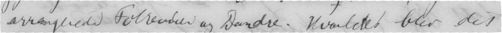arrangerede Folkeviser og Dandse. Hvorledes blev det
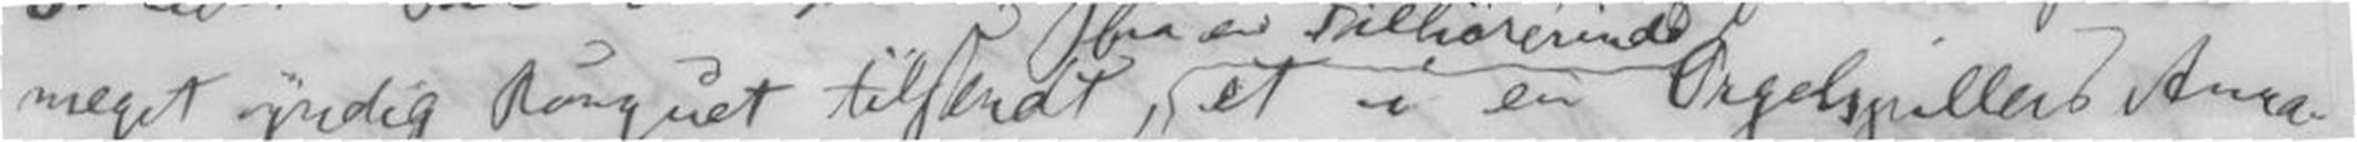meget yndig Bouquet tilsendt, et i en Orgelspillers Anna-
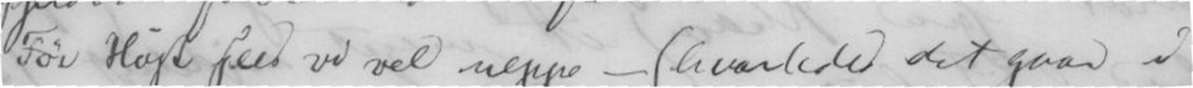Før Høst seer vi vel neppe - (hvorledes det gaar i
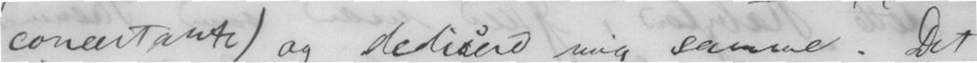concertante) og dedicere mig samme. Det
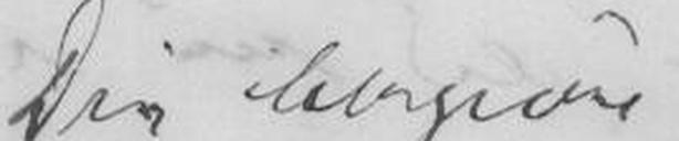Din hengivne
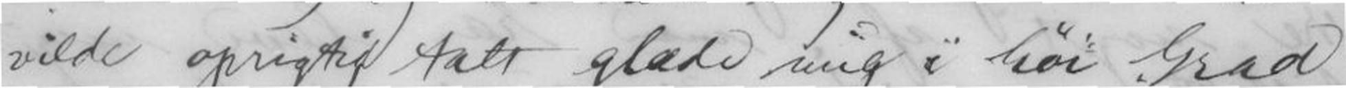vilde oprigtig talt glæde mig i høi Grad.
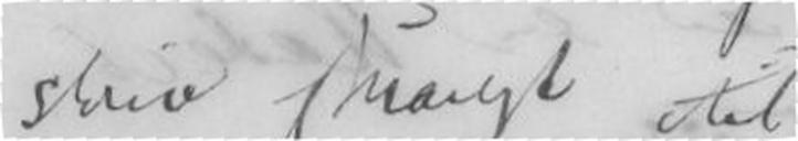skriv snarest til
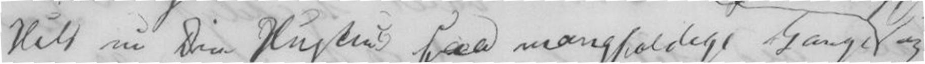Hils nu Din Hustru saa mangfoldige Gange og
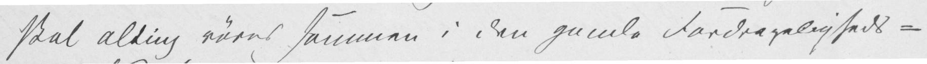skal alting røres sammen i den gamle Fordrageligheds-
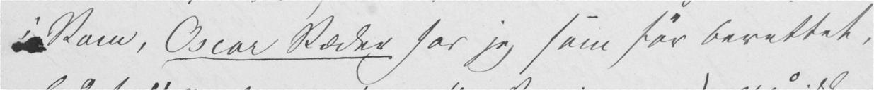i Rom, Oscar Ræder har jeg som før berettet,
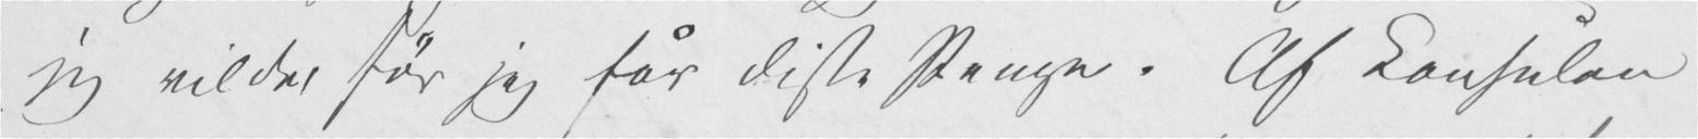jeg vilde før jeg får disse Penge. Af Konsulen
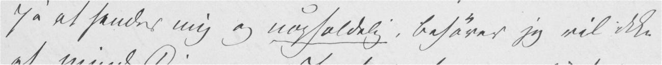på at sendes mig og uopholdelig, behøver jeg vil ikke
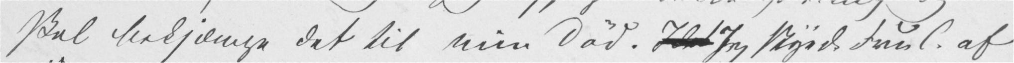skal bekjæmpe det til min Død. Idet Jeg skyede Fru C. af
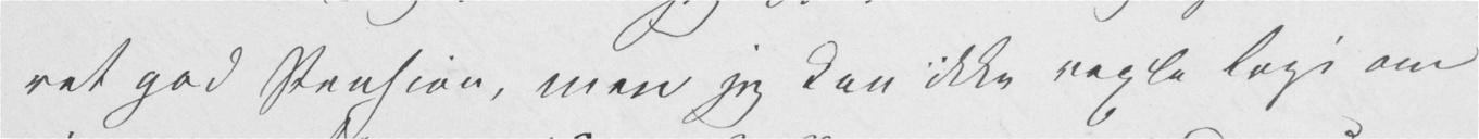ret god Pension, men jeg kan ikke vexle Logi om
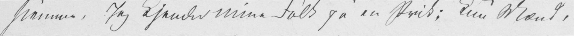hjemme, Jeg kjender mine Folk på en Prik; kun Mænd,
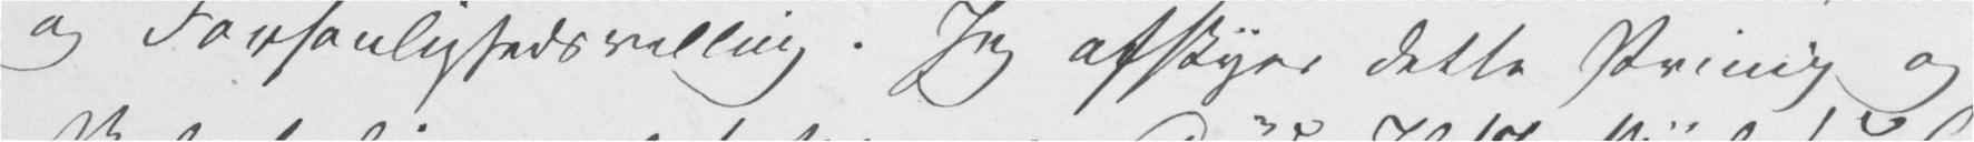og Forsonlighedsvelling. Jeg afskyer dette Princip og
